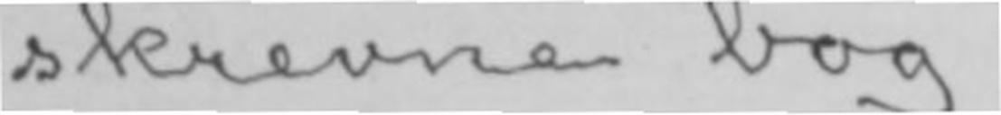skrevne bog.
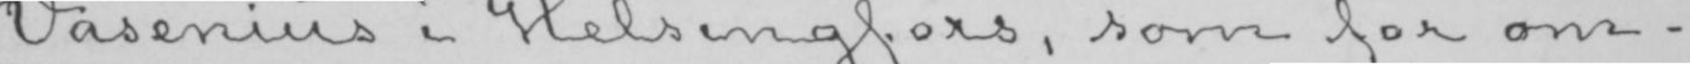Vasenius i Helsingfors, som for om-
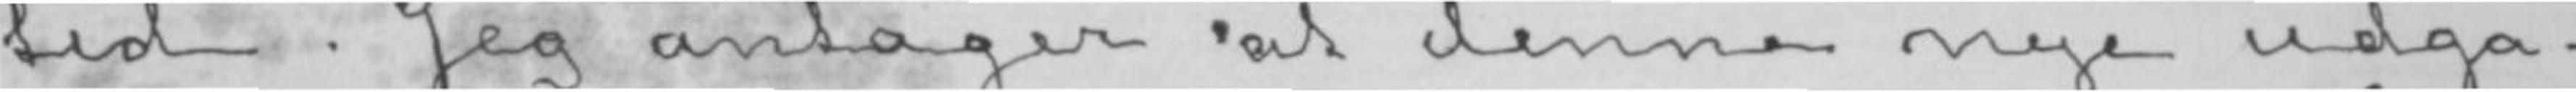tid. Jeg antager at denne nye udga-
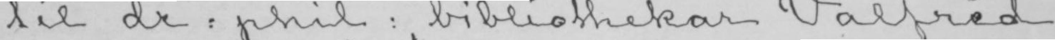til dr: phil:, bibliothekar Valfried
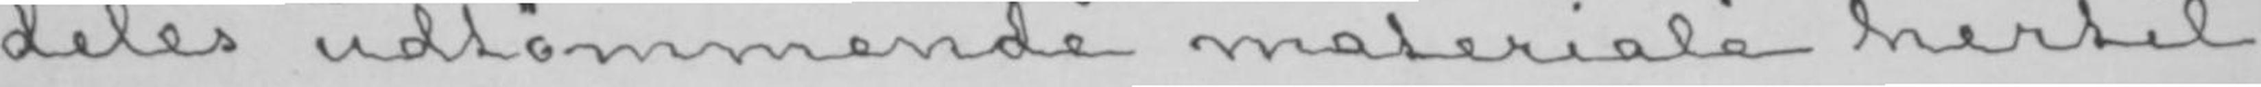deles udtømmende materiale hertil
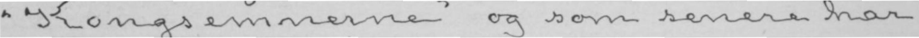«Kongsemnerne» og som senere har
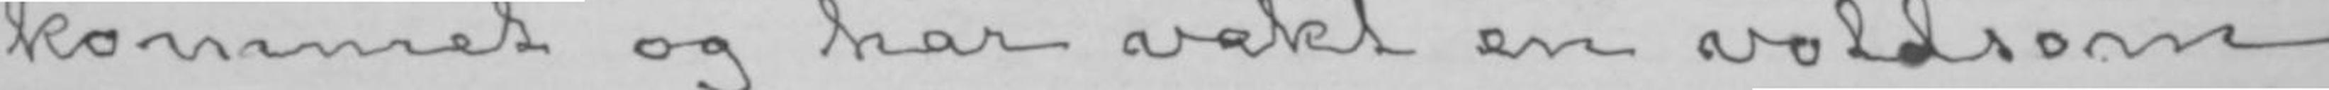kommet og har vakt en voldsom
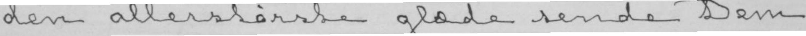den allerstørste glæde sende Dem
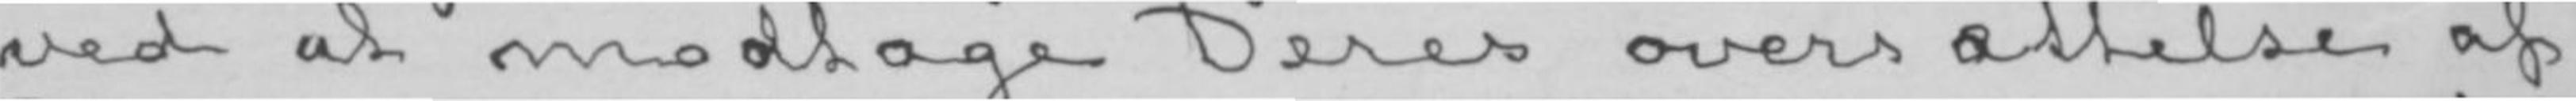ved at modtage Deres oversættelse af
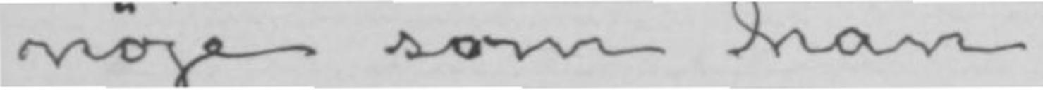nøje som han.
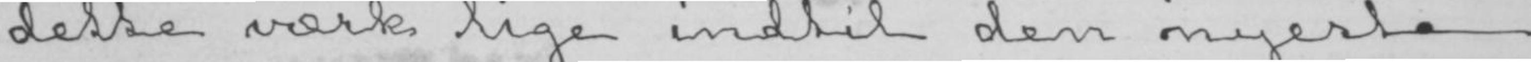dette værk lige indtil den nyeste
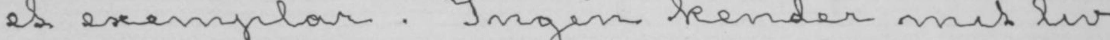et exemplar. Ingen kender mit liv
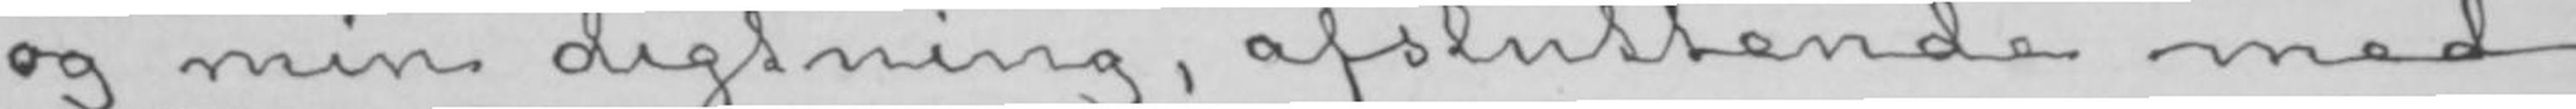og min digtning, afsluttende med
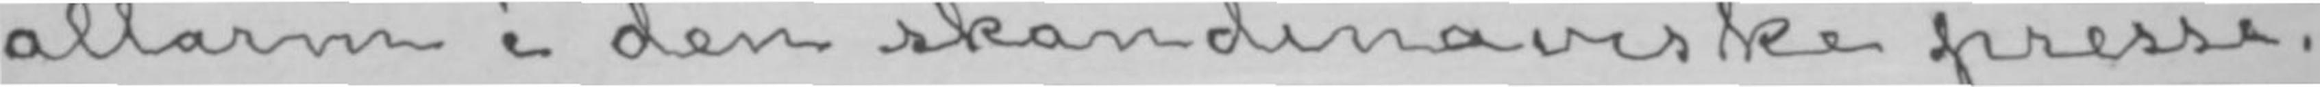allarm i den skandinaviske presse.
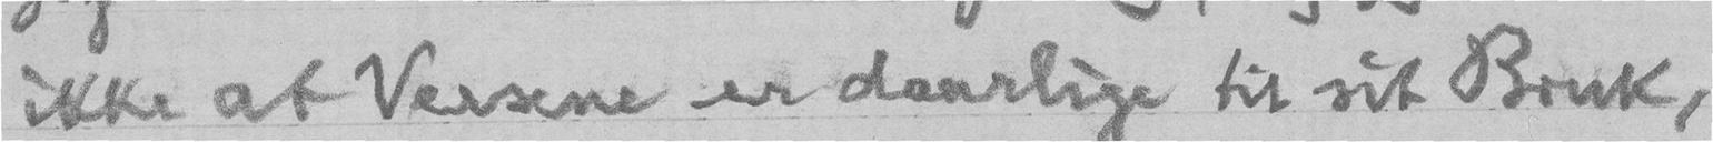ikke at Versene er daarlige til sit Bruk,
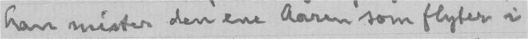han mister den ene Aaren som flyter i
